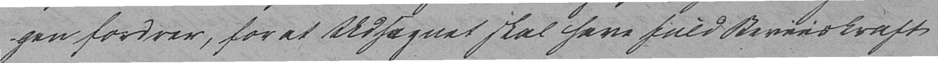gen fordrer, for at Udsagnet skal have fuld Beviiskraft
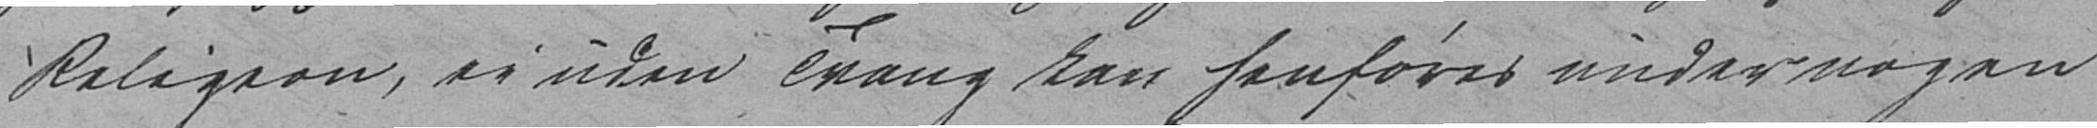Religion, ei uden Tvang kan henføres under nogen
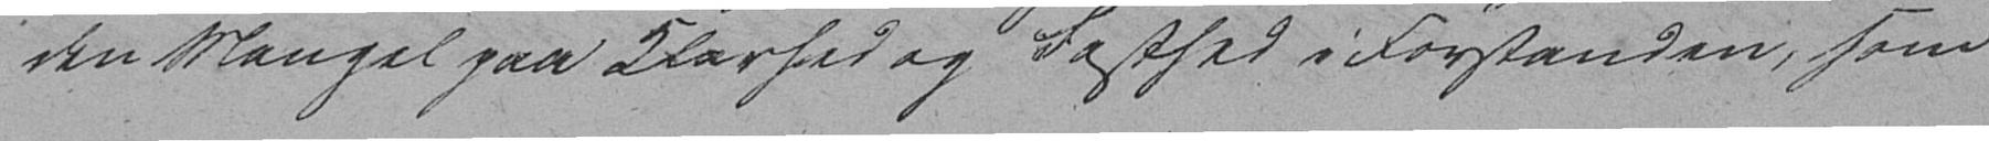den Mangel paa Klarhed og Fasthed i Forstanden, som
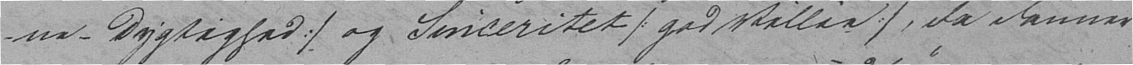ne - Dygtighed :/ og Sinceritet /: god Villie :/, da danner
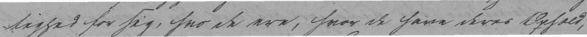tighed for sig, hvor de ere, hvor de have deres Ophold,
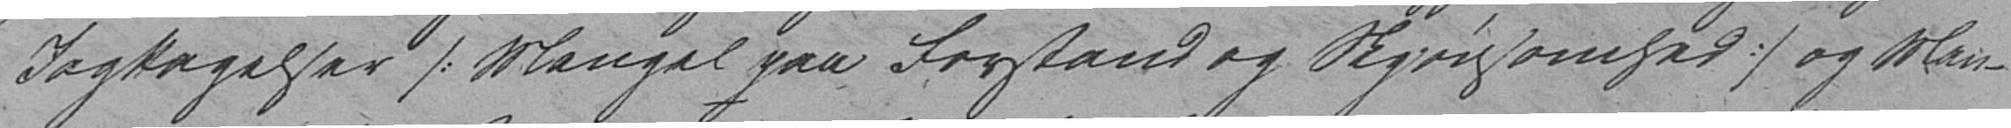Iagttagelser /: Mangel paa Forstand og Skjønsomhed :/ og Man-
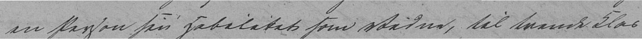en Person sin Habilitet som Vidne, til tvende Klas-
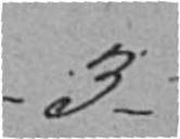- 3 -
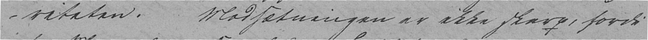riteten. Modsætningen er ikke skarp, fordi
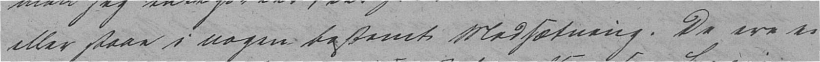eller staae i nogen bestemt Modsætning. De ere ei
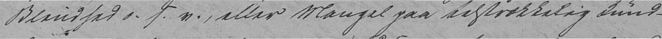Blindhed o. s. v., eller Mangel paa tilstrækkelig Kund-
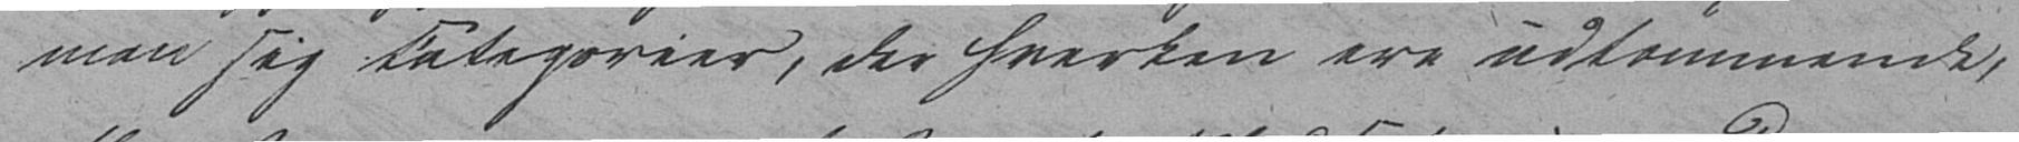man sig Categorier, der hverken ere udtømmende,
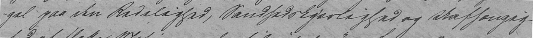gel paa den Redelighed, Sandhedskjærlighed og Uafhængig-
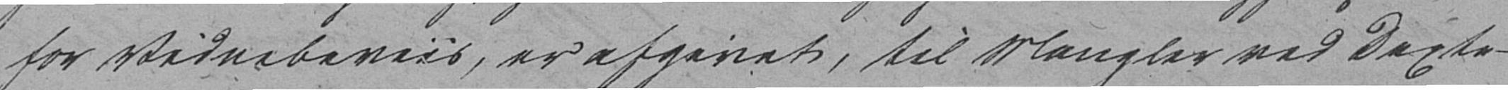for Vidnebeviis, er afgivet, til Mangler ved Dexte-
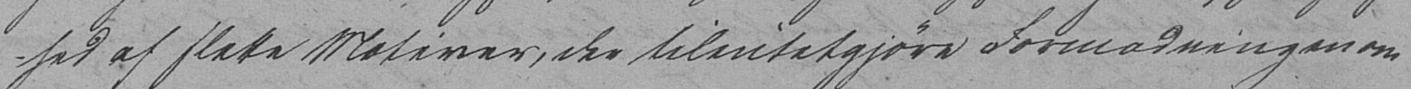hed af slette Motiver, der tilintetgjøre Formodningen om
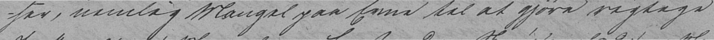ser, nemlig Mangel paa Evne til at gjøre rigtige
